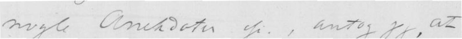nogle Anekdoter osv., antog jeg, at
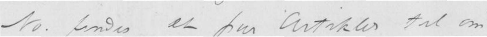No. findes et par Artikler til om
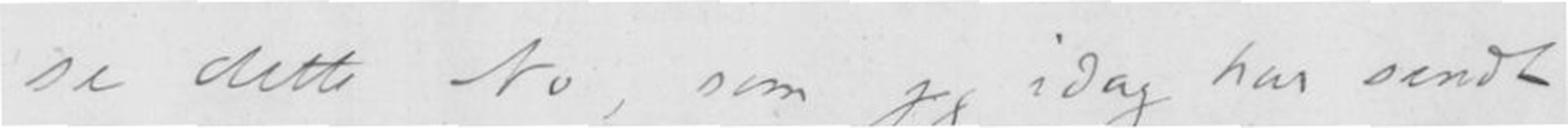se dette No, som jeg idag har sendt
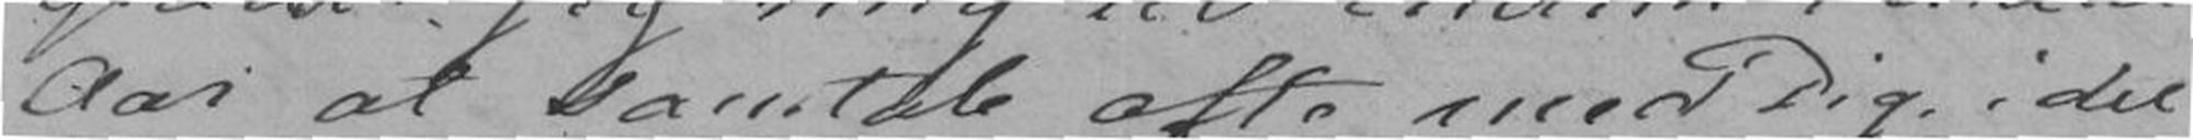Aar at samtale ofte med Dig i det
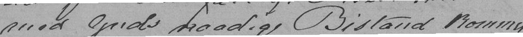med Guds naadige Bistand kommer
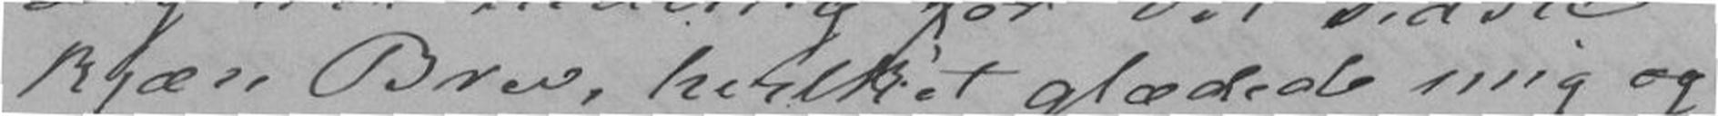kjære Brev, hvilket glædede mig og
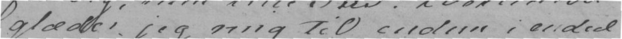glæder jeg mig til endnu i endeel
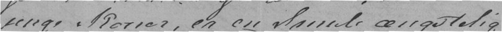unge Koner, er en Smule ængstelig
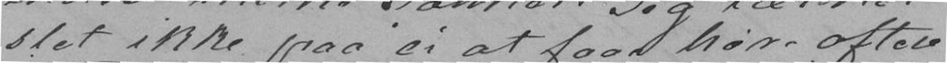slet ikke paa ei at faae høre oftere
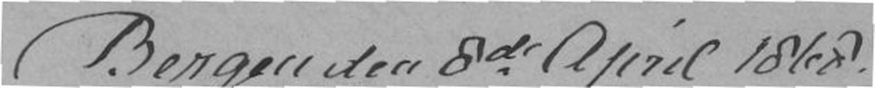Bergen den 8de April 1868.
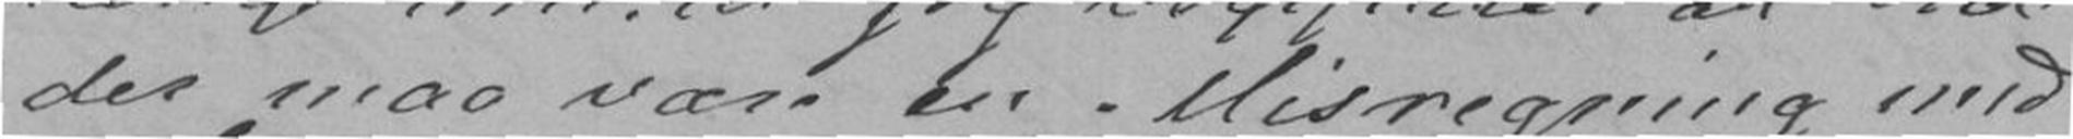der maa være en Misregning med
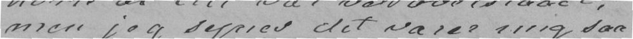men jeg synes det varer mig saa
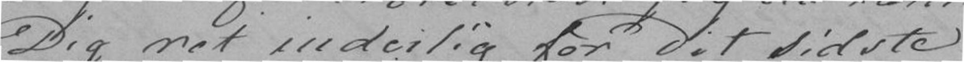Dig ret inderlig for Dit sidste
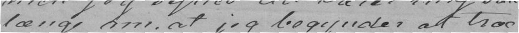længe nu, at jeg begynder at troe
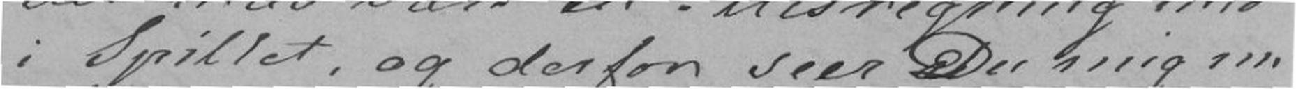i Spillet, og derfor seer Du mig nu
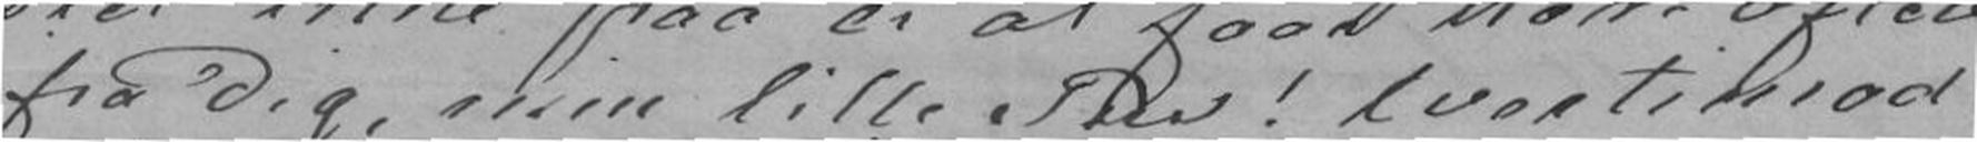fra Dig, min lille Pus! tvertimod
